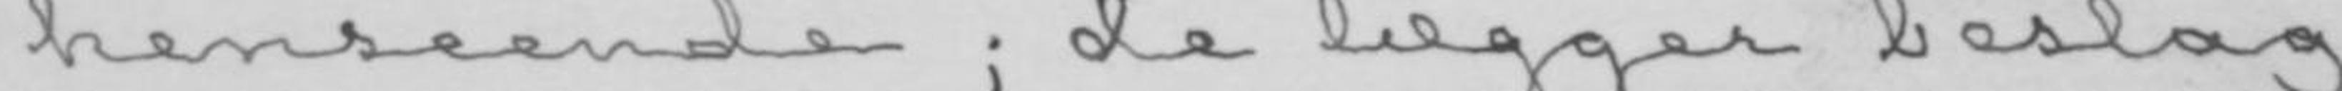henseende; de lægger beslag
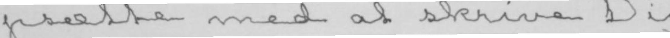opsætte med at skrive Dig
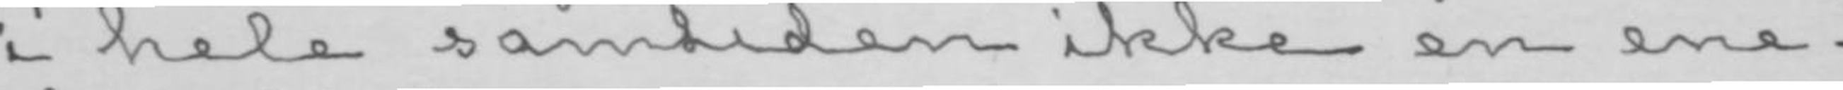i hele samtiden ikke en ene-
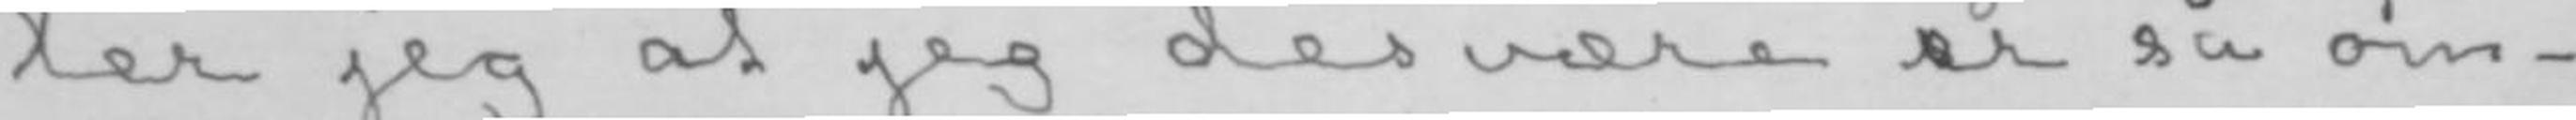ler jeg at jeg desvære er så om-
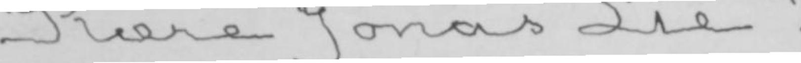Kære Jonas Lie!
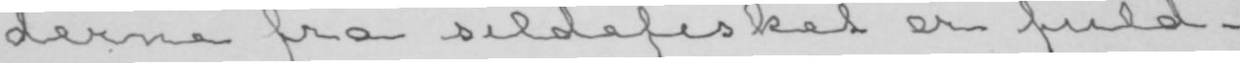derne fra sildefisket er fuld-
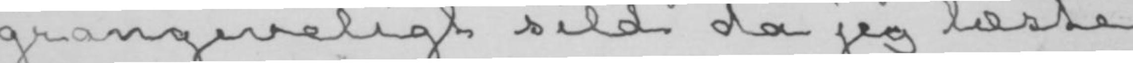grangiveligt sild da jeg læste
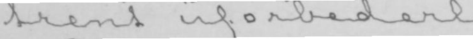trent uforbederlig.
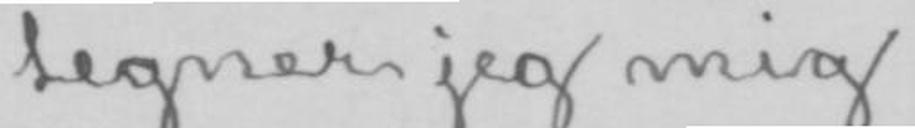tegner jeg mig
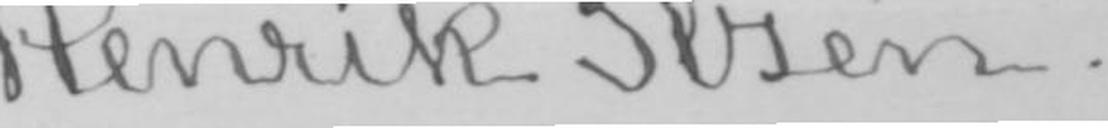Henrik Ibsen.
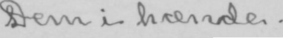Dem i hænde.
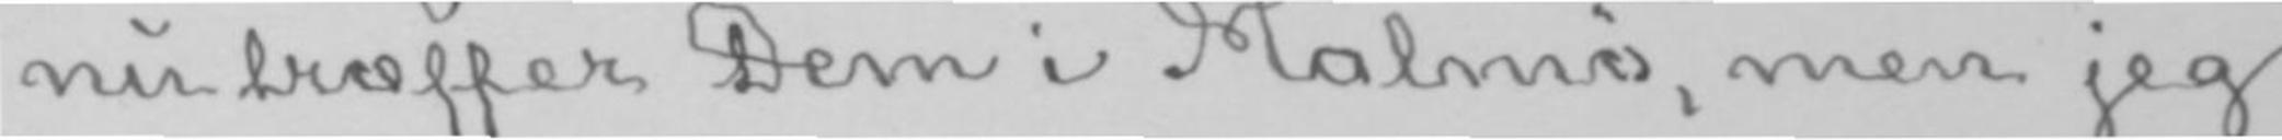nu træffer Dem i Malmö, men jeg
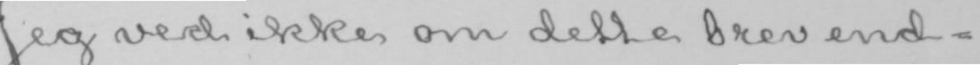Jeg ved ikke om dette brev end-
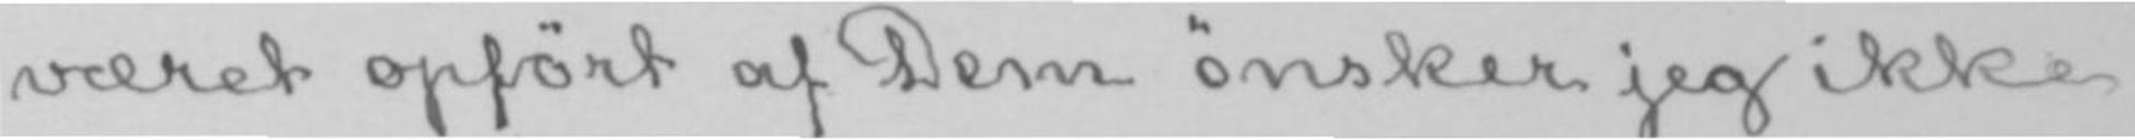været opført af Dem ønsker jeg ikke
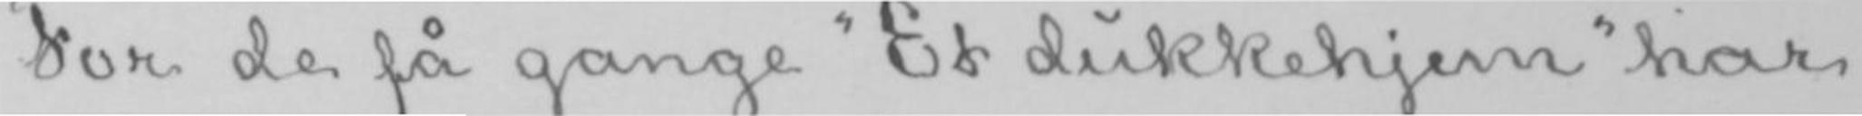For de få gange «Et dukkehjem» har
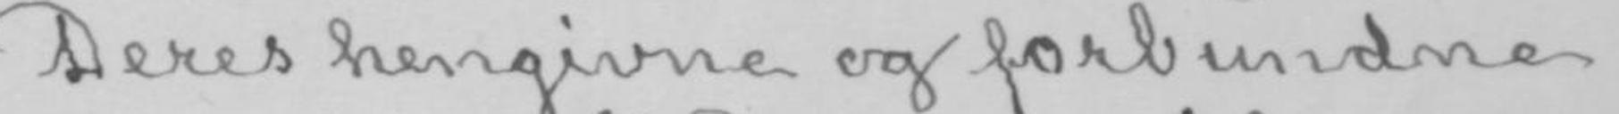Deres hengivne og forbundne
